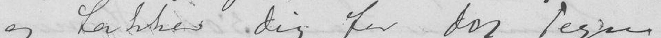og takker Dig for Dit Tegn
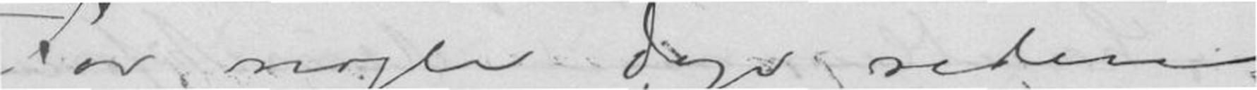For nogle Dage siden
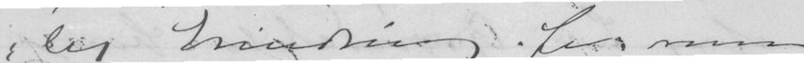lig Erindring for mine
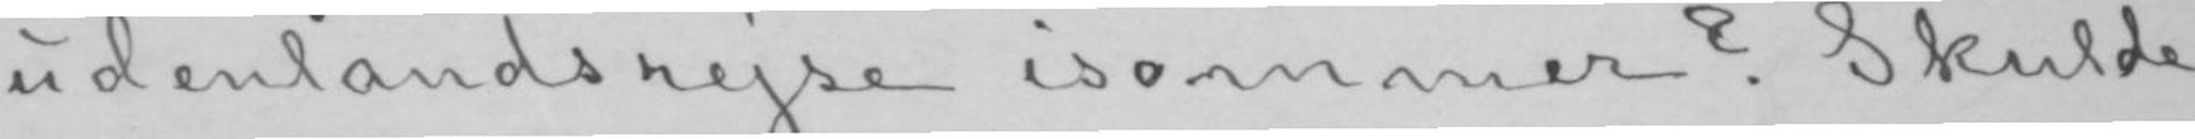udenlandsrejse isommer? Skulde
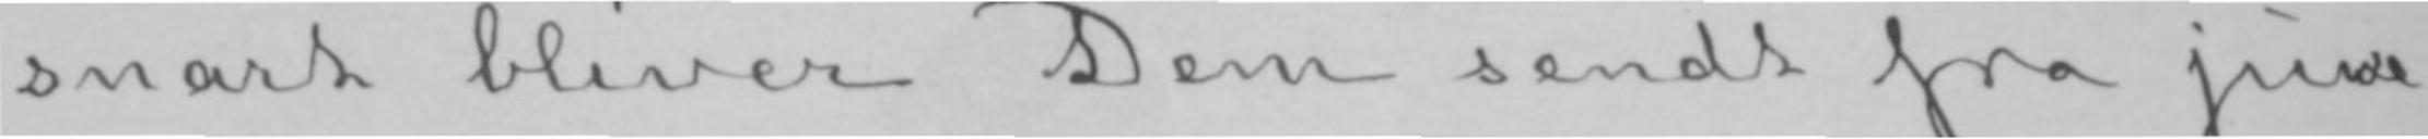snart bliver Dem sendt fra juve-
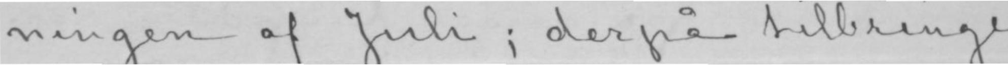ningen af Juli; derpå tilbringer
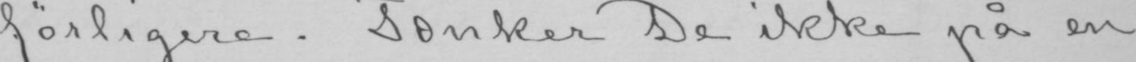førligere. Tænker De ikke på en
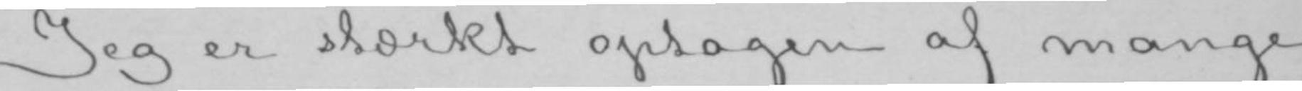Jeg er stærkt optagen af mange-
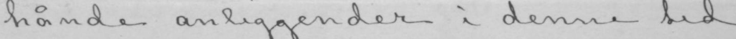hånde anliggender i denne tid
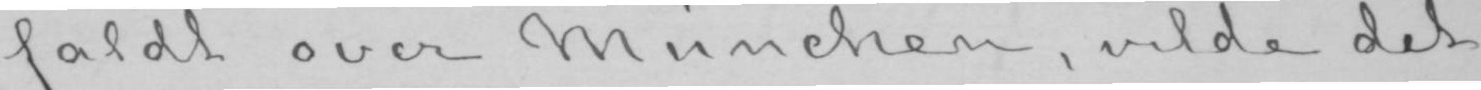faldt over München, vilde det
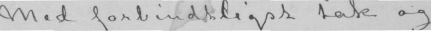Med forbindtligst tak og
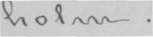holm.
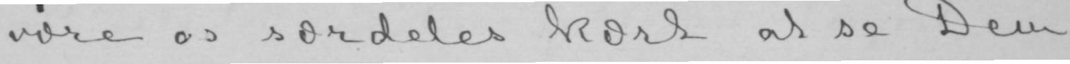være os særdeles kært at se Dem
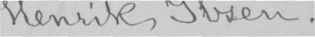Henrik Ibsen.
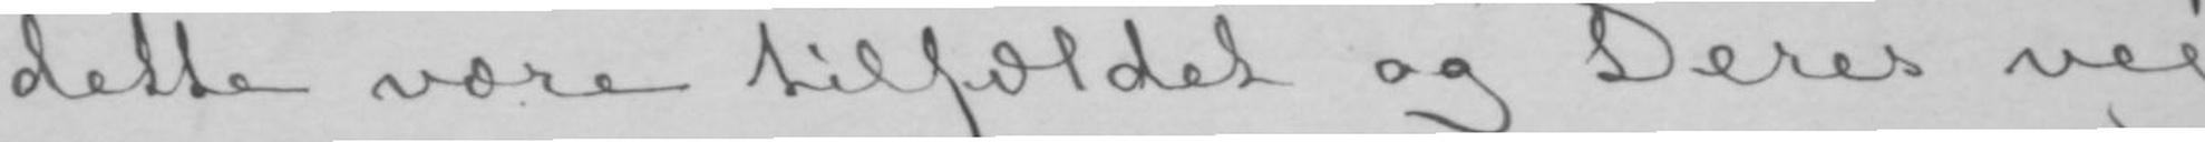dette være tilfældet og Deres vej
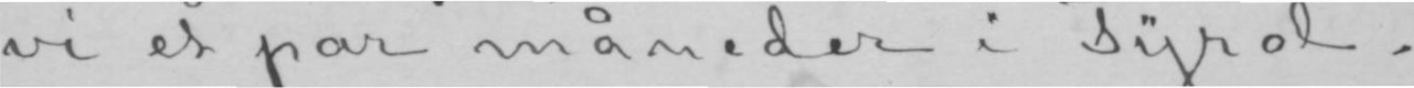vi et par måneder i Tyrol.
